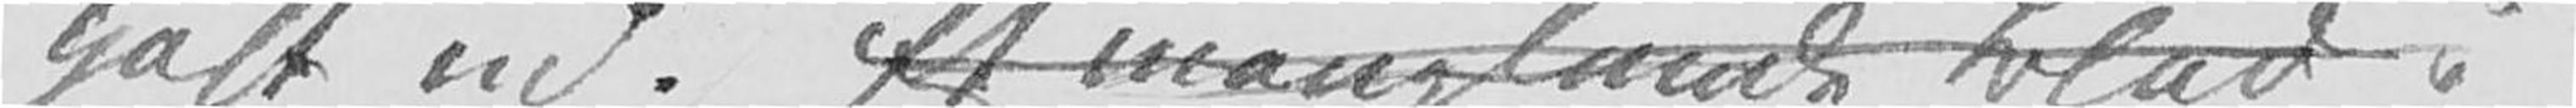galt ud. Et manglende Blad i
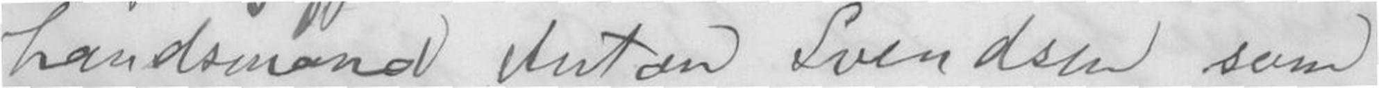Landsmand Anton Svendsen som
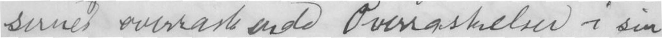sernes overraskende Overraskelser i sin
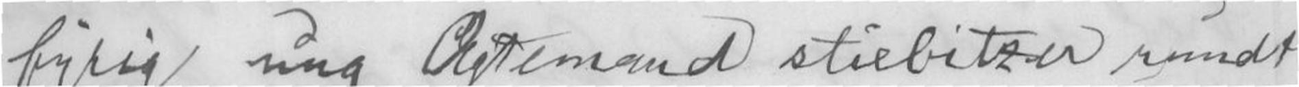fyrig ung Ægtemand stiebitzer rundt
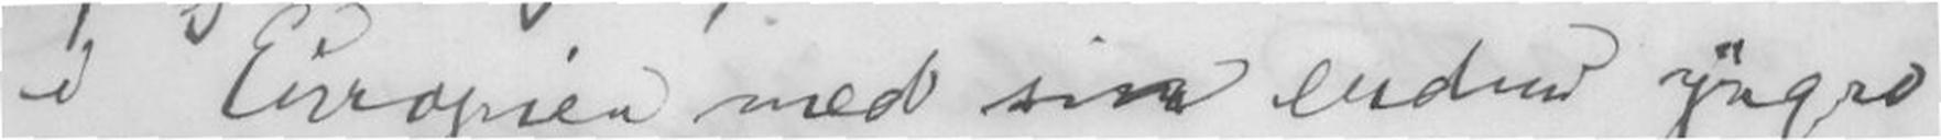i Europien med sin endnu yngre
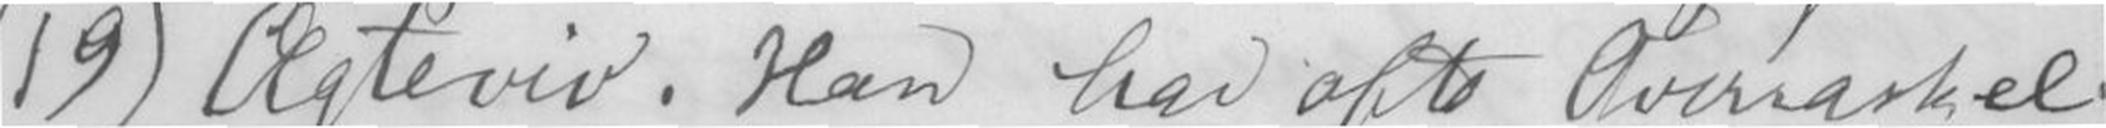(19) Ægteviv. Han har ofte Overraskel-
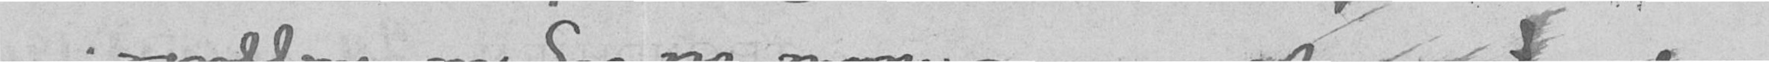meget av det. Gid det ikke måtte bli dig en skuffelse!
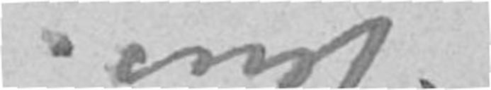Jens
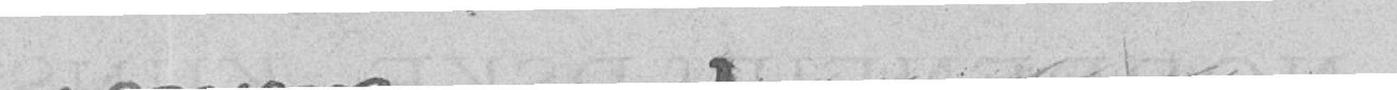Levvel kjære Gustav!
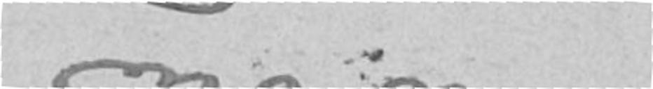Din ven
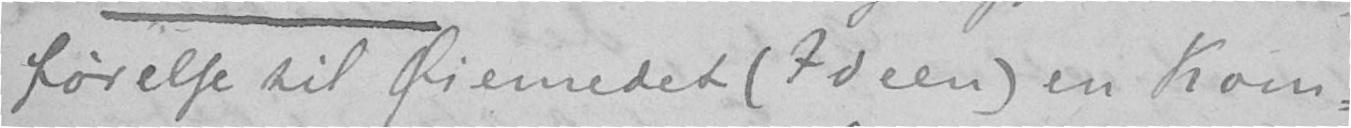førelse til Øiemedet (Ideen) en Kom-
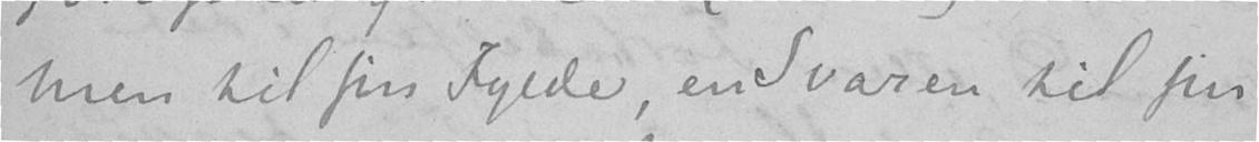men til sin Fylde, en Svaren til sin
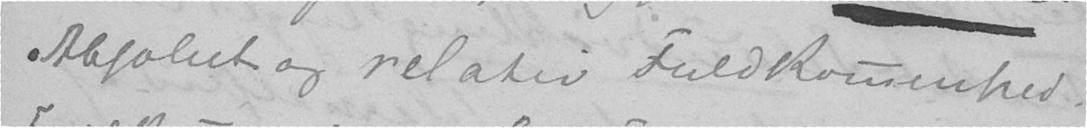Absolut og relativ Fuldkommenhed.
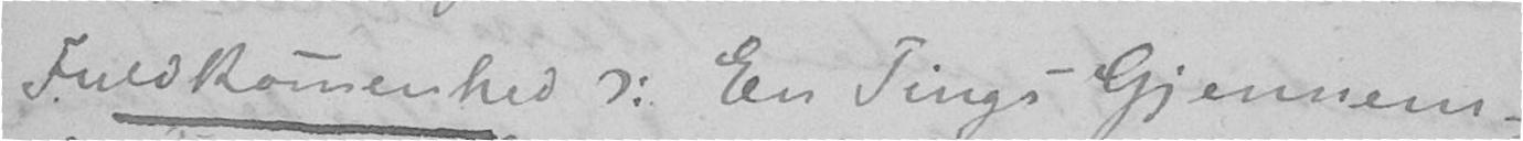Fuldkommenhed ): En Tings Gjennem-
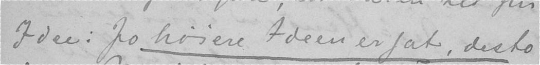Idee: Jo høiere Ideen er sat, desto
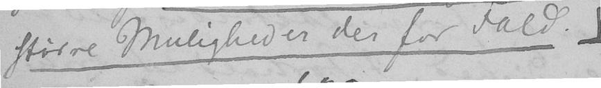større Mulighed er der for Fald.
